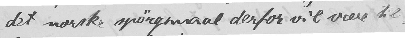det norske spørgsmaal derfor vil være til-
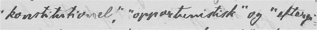"konstitutionel", "opportunistisk" og "eftergi-
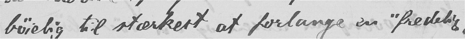bøielig til stærkest at forlange en "fredelig",
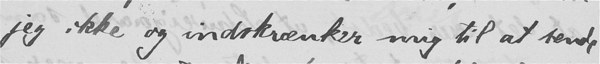jeg ikke og indskrænker mig til at sende
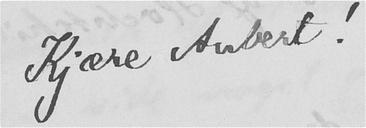Kjære Aubert!
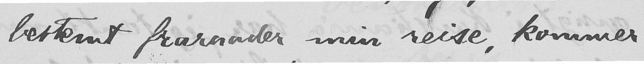bestemt fraraader min reise, kommer
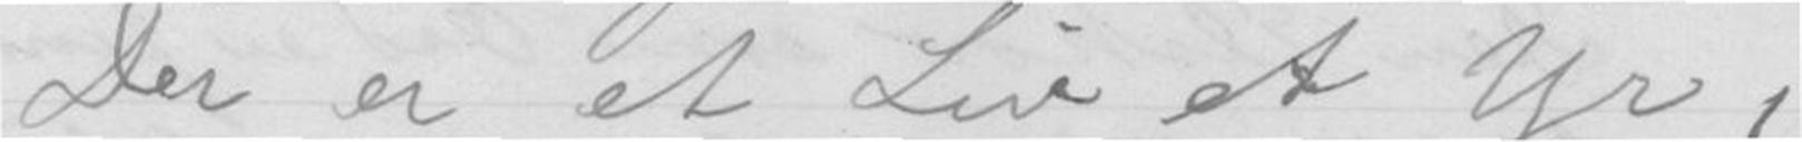Der er et Liv et Yr,
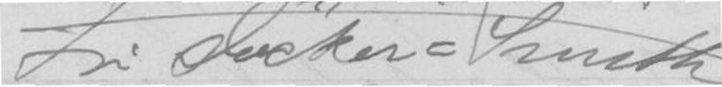Fr. Dösker-Smith
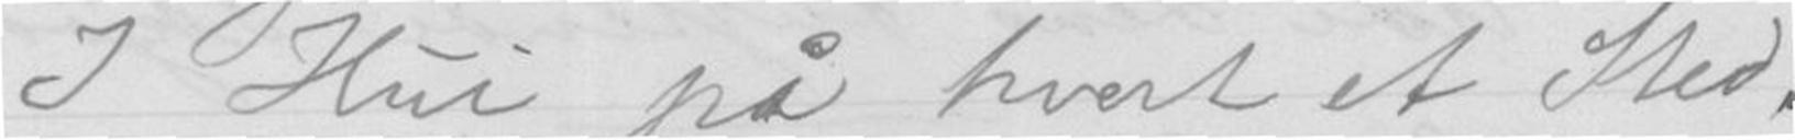I Hui på hvert et Sted.
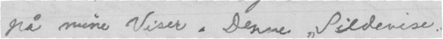på mine Viser. Denne "Sildevise
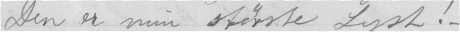Den er min største Lyst! -
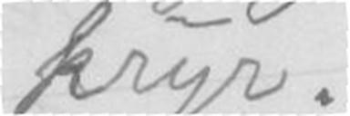kryr.
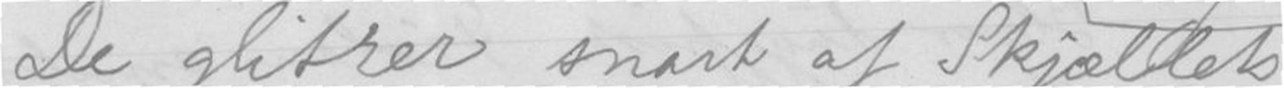De glitrer snart af Skjællets
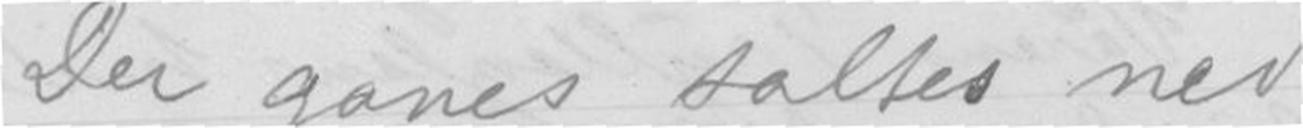Der ganes saltes ned
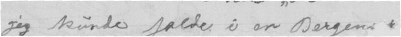jeg kunde falde i er Bergens
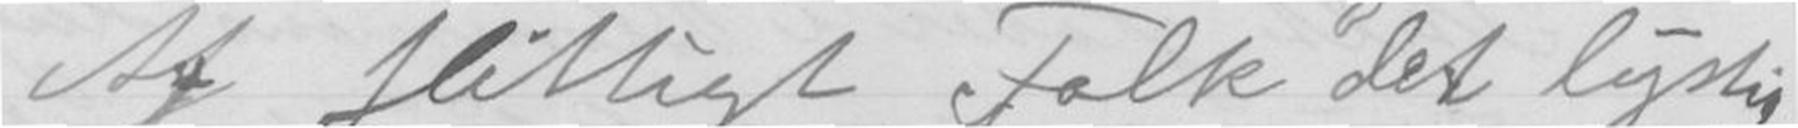Af flittigt Folk det lystig
